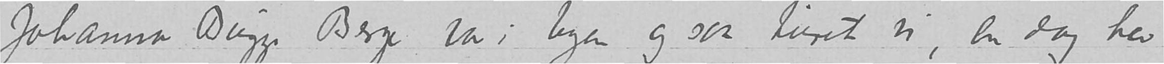Johanna Bugge Berge var i byen og saa turet vi, en dag her
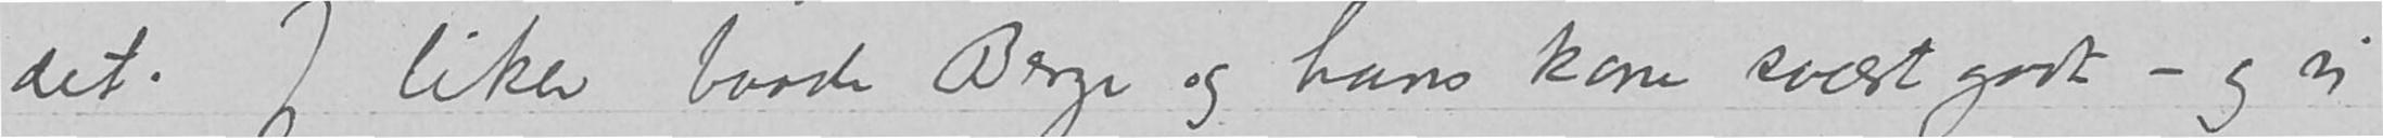det. Jeg liker baade Berge og hans kone svært godt -og vi
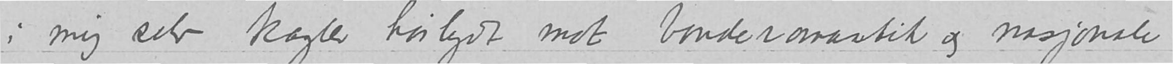i mig selv koagler høilydt mot bonderomantik og nasjonale rørelser
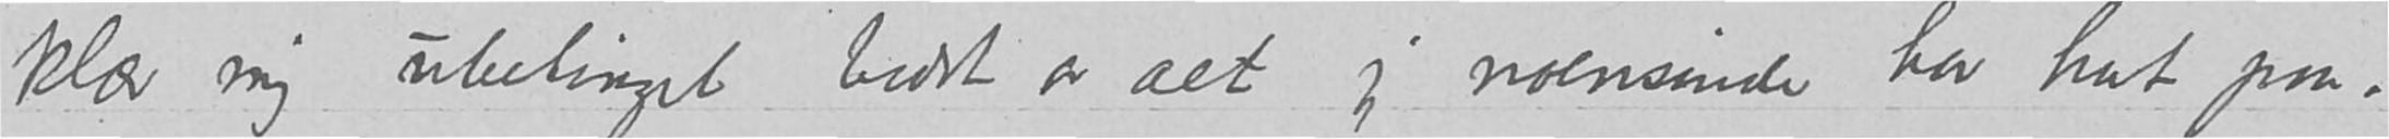klær mig ubetinget bedst av alt jeg noensinde har hat paa.
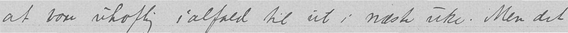at være uhøflig ialfald til ut i næste uke. Men det
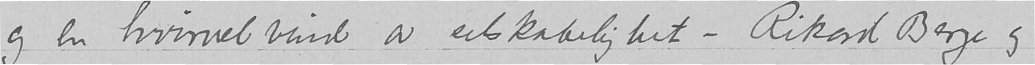og en hvirvelvind av selskabelighet -Rikard Berge og
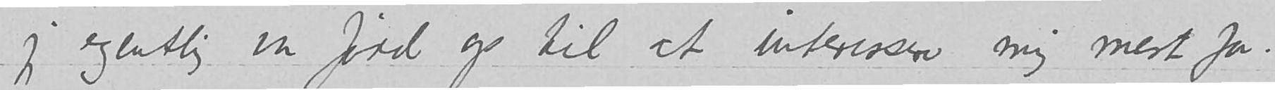jeg egentlig vaer fødd op til at interessere mig mest for.
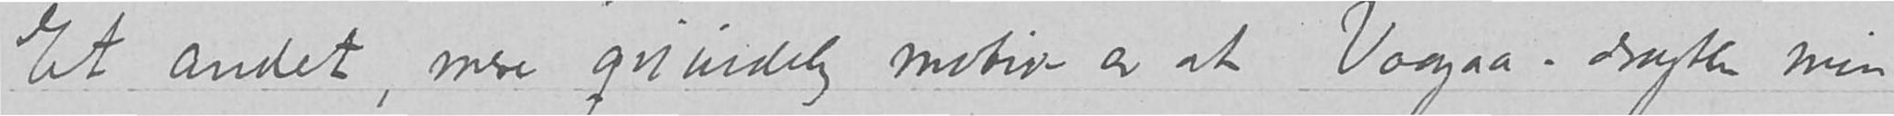Et andet, mere qvindelig motiv er at Vaagaa-dragten min
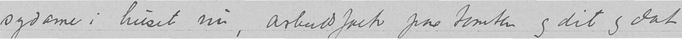sydame i huset nu, arbeidsfolk paa tomten og dit og dat
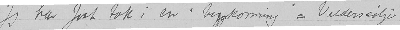Jeg har faat tak i en «byggkornring» = Valderssølje
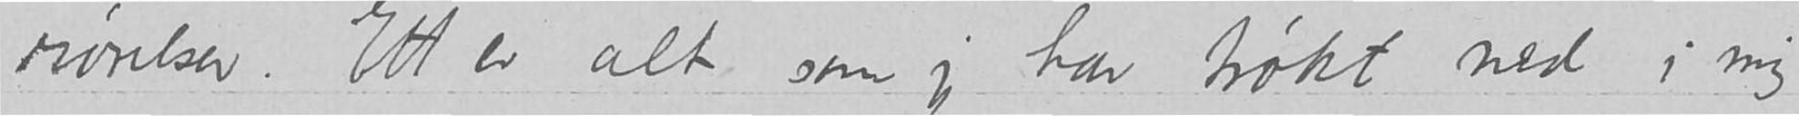Ett er alt som jeg har trøkt ned i mig
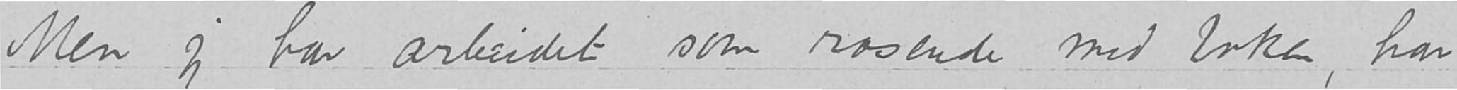Men jeg har arbeidet som rasende med boken, har
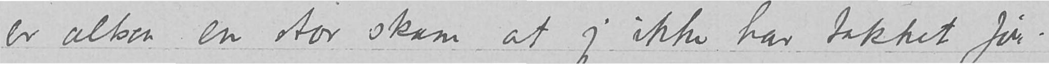er altsaa en stor skam at jeg ikke har takket før.
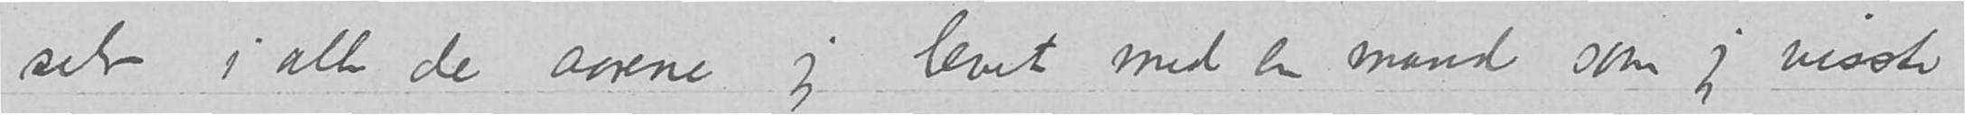selv i alle de aarene jeg levet med en mand som jeg visste
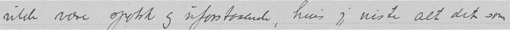vilde være spotsk og uforstaaende, hvis jeg viste alt det som
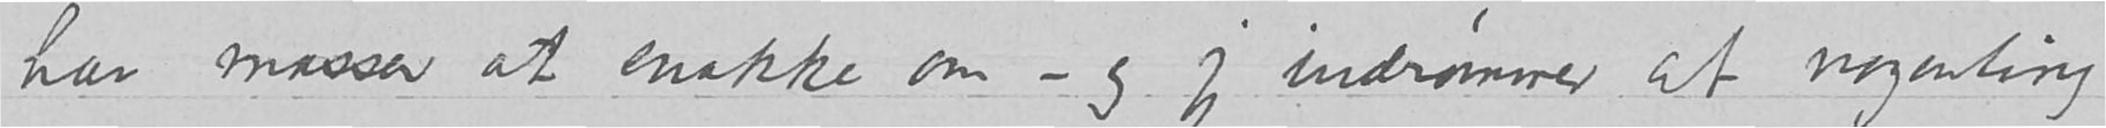har masser at snakke om -og jeg indrømmer at nogenting
